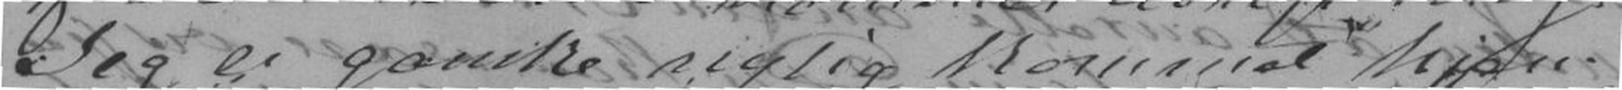Jeg er ganske rigtig kommet hjem
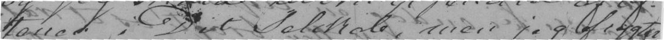tene i dit Selskab, men jeg frygter
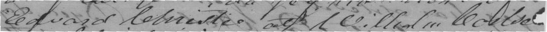Edvard Christie at Wilhelm Coilse
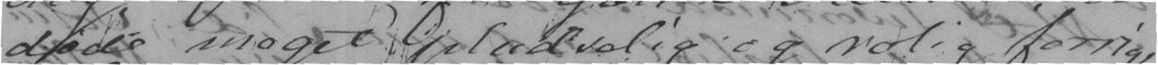døde meget pludselig og rolig forrige
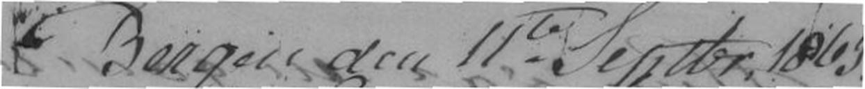Bergen den 11te Septbr. 1865.
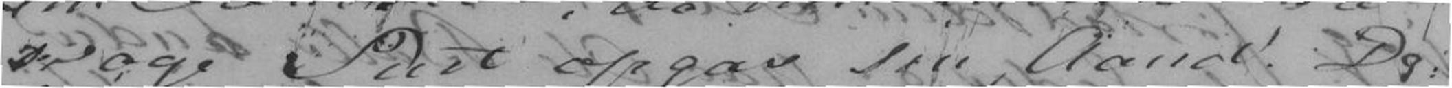svage Pust opgav sin Aand. De
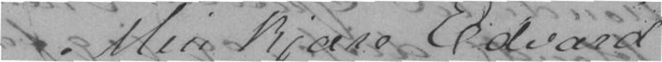Min kjære Edvard!
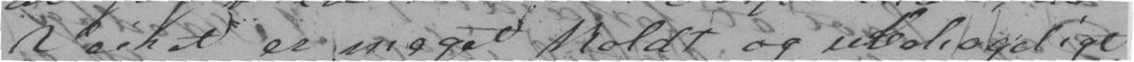Veiret er meget koldt og ubehageligt,
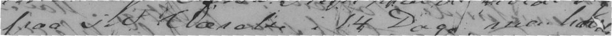paa sit Værelse i 14 Dage, men have
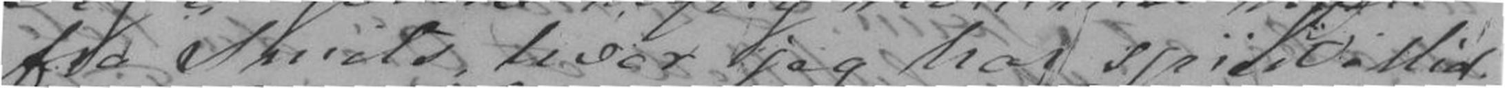fra Smits hvor jeg har spiist Mid-
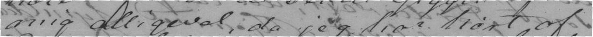mig alligevel, da jeg har hørt af
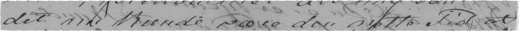det nu kunde være den rette Tid at
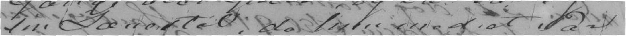sin Lænestol; der hun med et Par
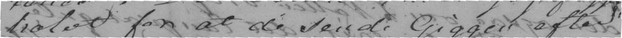halvt for at de sende Giggen efter
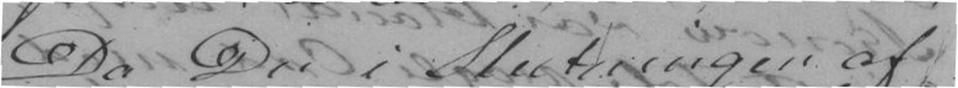Da Du i Slutningen af
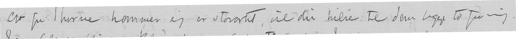At fru Thorne kommer sig er storartet, vil du hilse til dem begge to fra mig.
